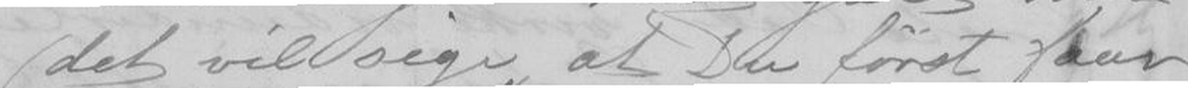(det vil sige, at Du først faar
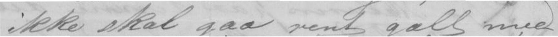ikke skal gaa rent galt med
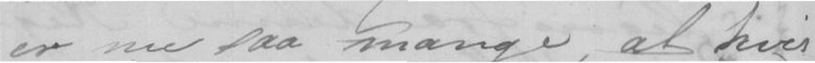er nu saa mange, at hvis
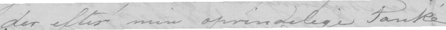der efter min oprindelige Tanke
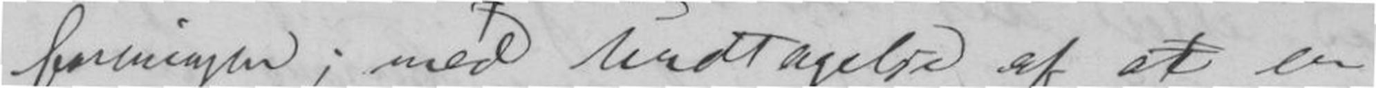foreningen; med Undtagelse af at en
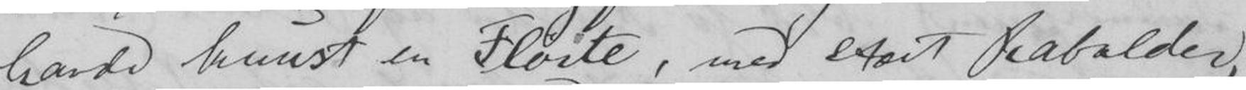havde kunst en Fløite, med stort Rabalder,
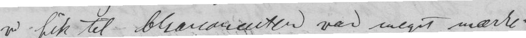vi fik til Charconcerten var meget mærke-
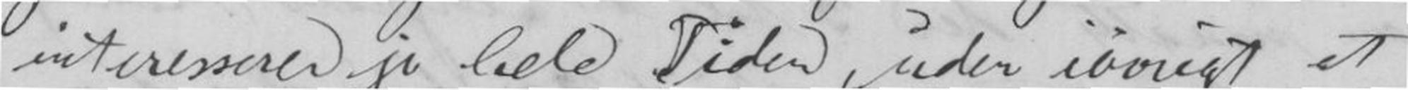intersserer jo hele Tiden uden iøvrigt at
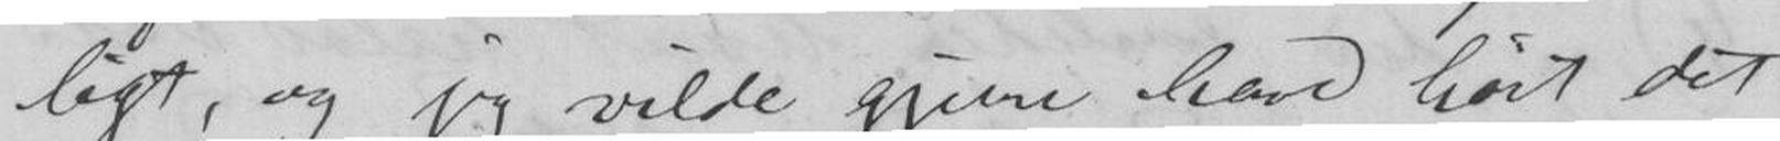ligt, og jeg vilde gjerne have hørt det
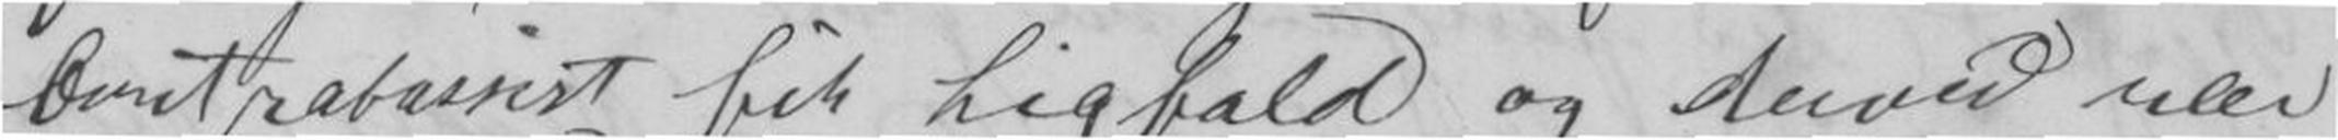Contrabassist fik Ligfald og derved nær
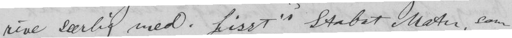rive særlig med. Liszt`s Stabat Mater, som
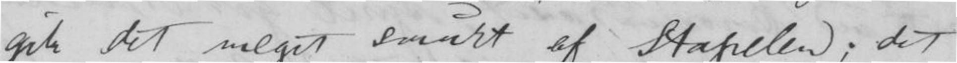gik det meget smurt af Stapelen; det
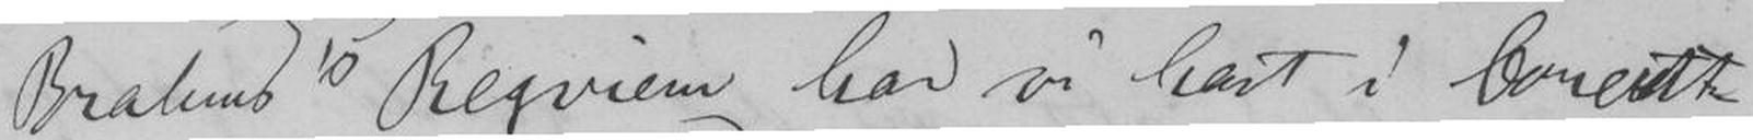Brahms's. Reqviem har vi havt i Concert
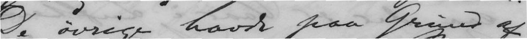De øvrige havde paa Grund af
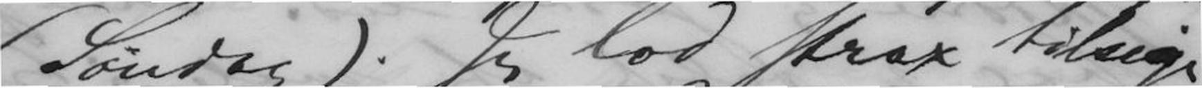(Søndag). Jeg lod strax tilsige
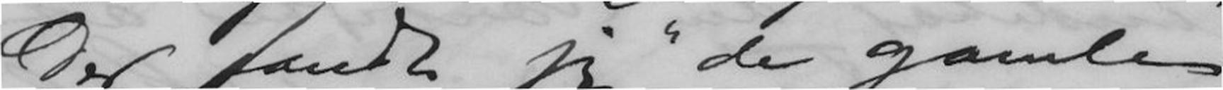Der fandt jeg "de gamle
